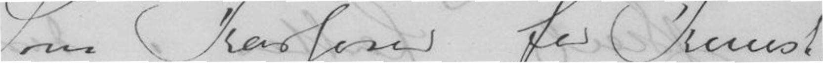Som Kasserer for Kunst-
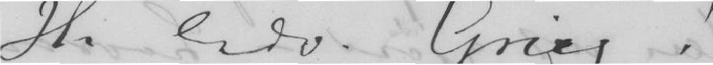Hr Edv. Grieg!
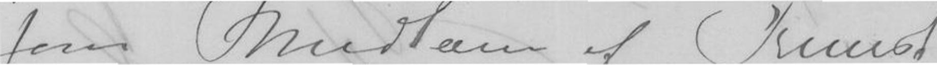som Medlæm af Kunst-
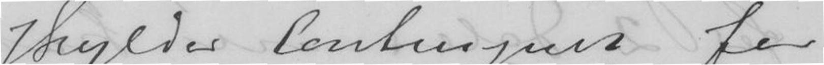skylder Contingent for
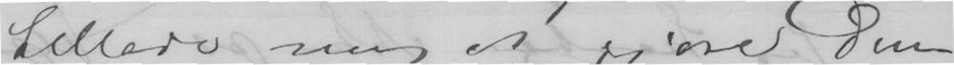tillade mig at gjøre Dem
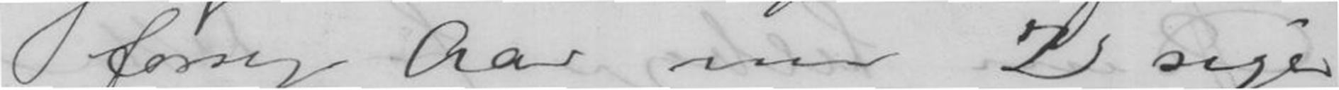forrig Aar nu 2 siger
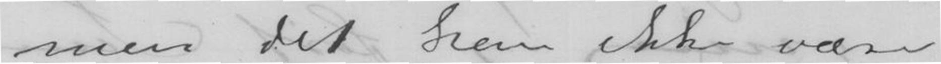men det kan ikke være
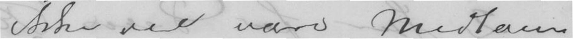ikke vil være Medlæm
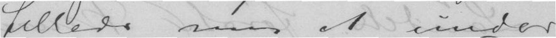tillade mig at under-
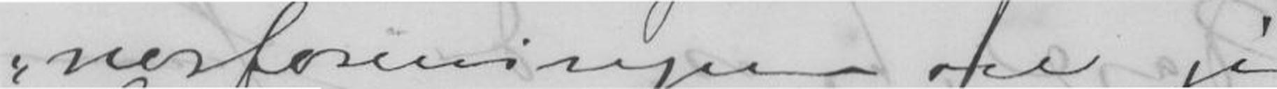nerforeningen vil jeg
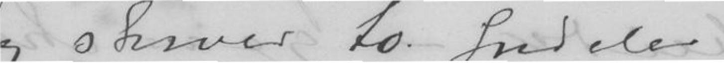og skriver to spdaler.
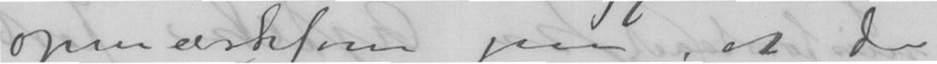opmærksom paa at de
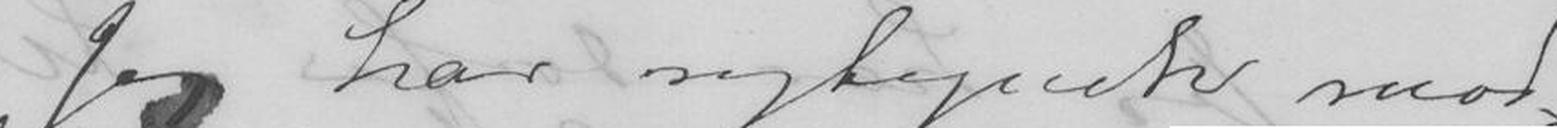Jeg har rigtignok mod-
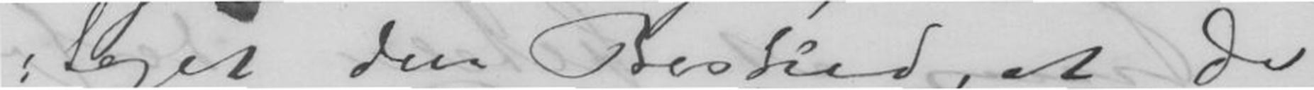taget den Besked, at De
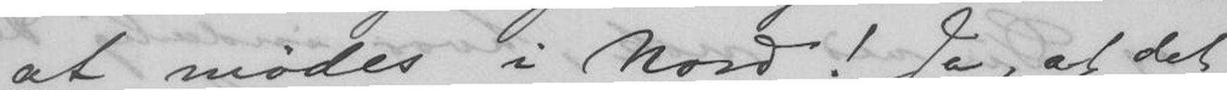at mødes i Nord! Ja, at det
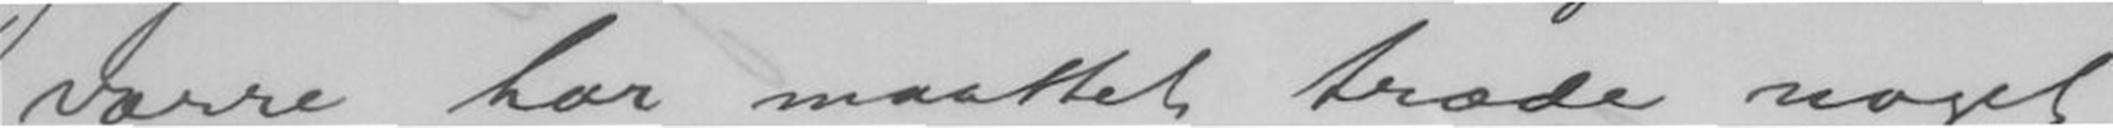værre har maattet træde noget
